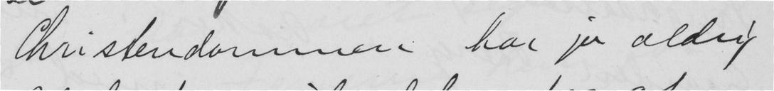Christendommen har jo aldrig
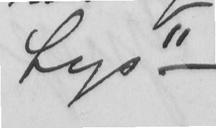lys" -
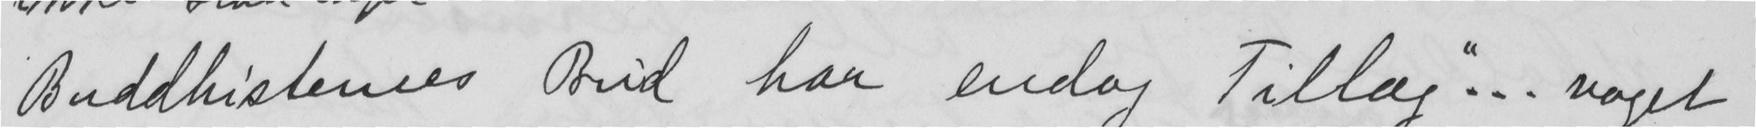Buddhistenes Bud har endog Tillag "...noget
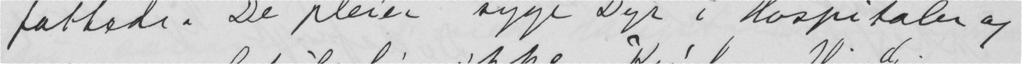fattede. De pleier syge Dyr i Hospitaler og
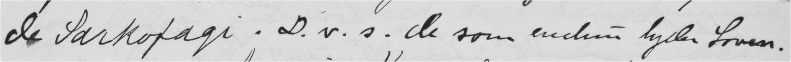de Sarkofagi. D. v. s. de som endnu lyder Loven.
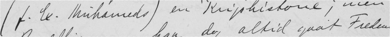(f. ex. Muhameds) en Krigshistorie; men
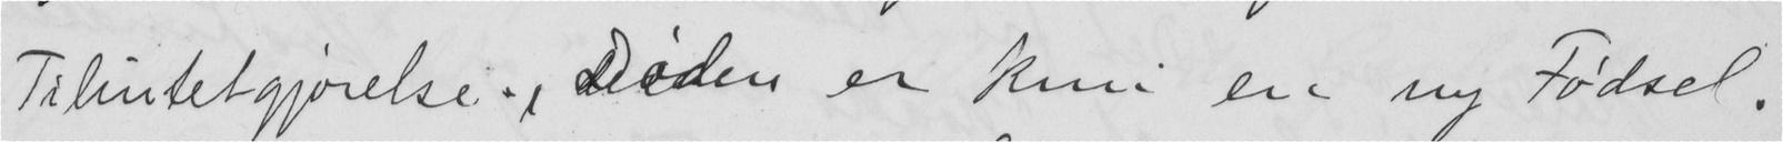Tilintetgjørelse. Daden er kun en ny Fødsel.
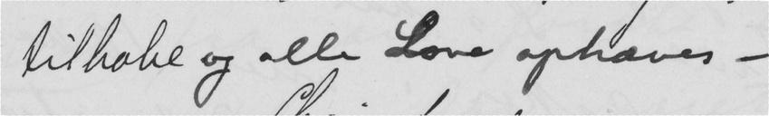tilbake og alle Love ophæves -
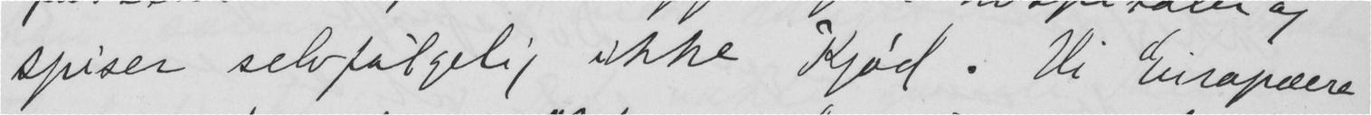spiser selvfølgelig ikke Kjød. Vi Europæere
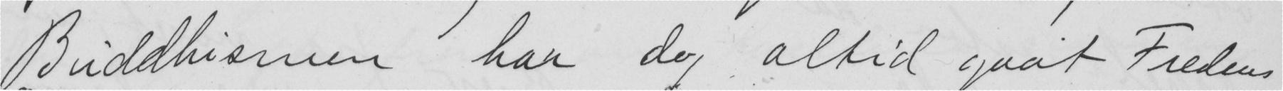Buddhismen har dog altid gaat Fredens
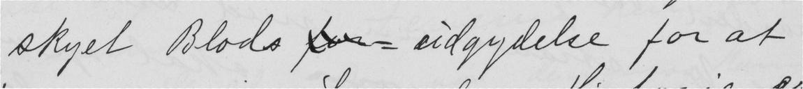skyet Blods  udgydelse for at
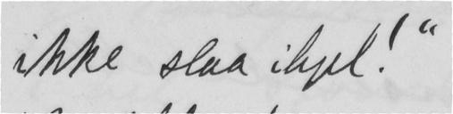ikke slaa ihjel!"
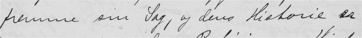fremme sin Sag, og dens Historie er
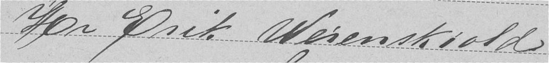Hr Erik Werenskiold
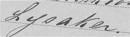Lysaker
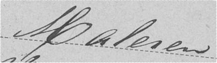Maleren
